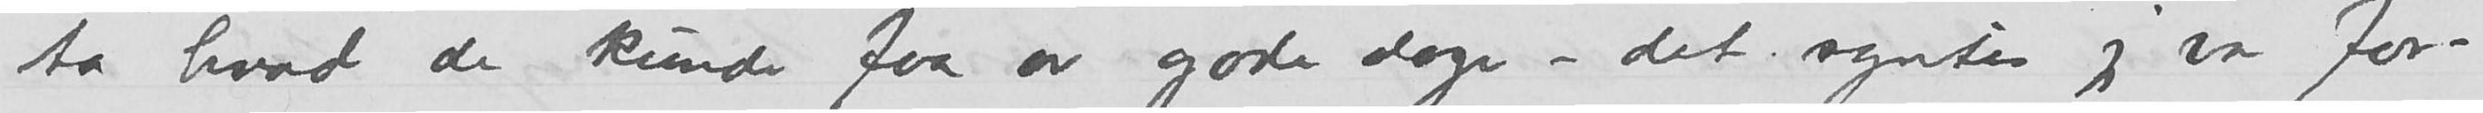ta hvad de kunde faa av gode dager -det syntes jeg var for
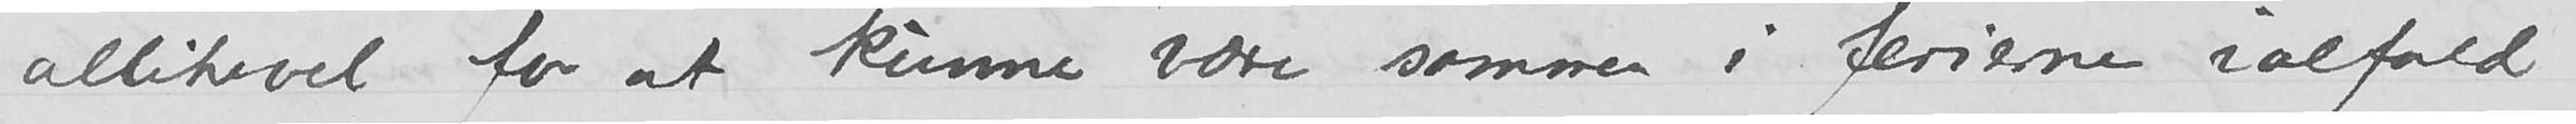allikevel for at kunne være sammen i ferierne ialfald.
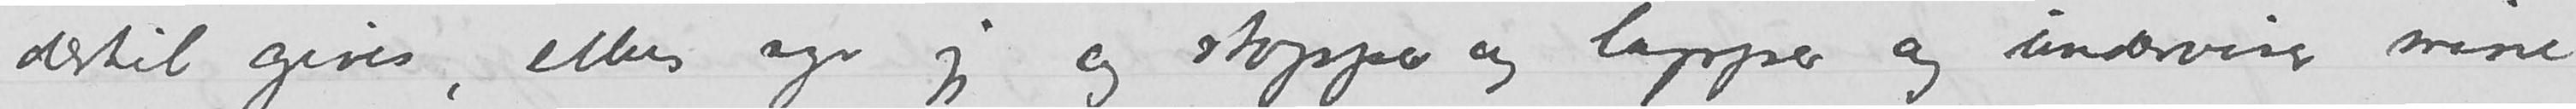dertil gives, ellers syr jeg og stopper og lapper og underviser mine
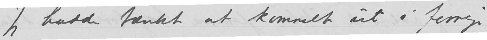Jeg hadde tænkt at kommet ut i forrige
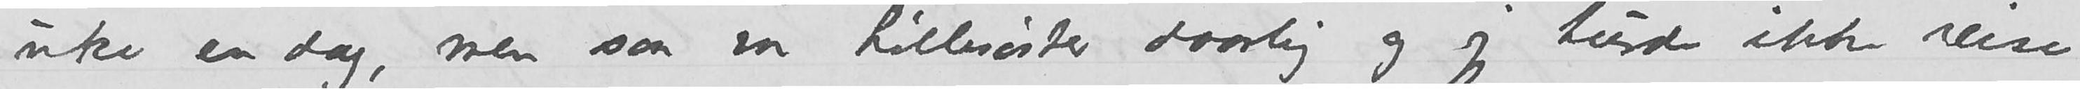uke en dag, men saa var er Lillesøster daarlig og jeg turde ikke reise
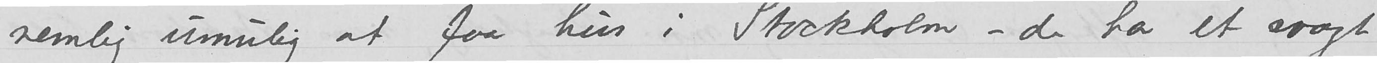nemlig umulig at faa hus i Stockholm -de har et svagt
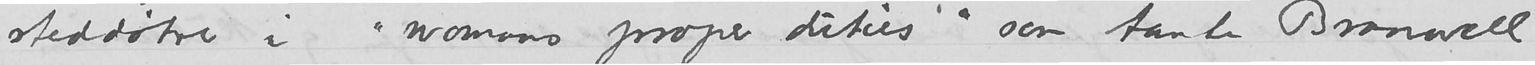steddøtre i «womans proper duties» som tante Branwell
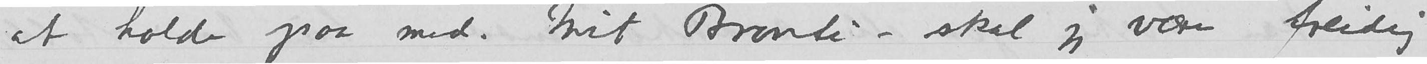at holde paa med. Mit Brontë - skal jeg være freidig
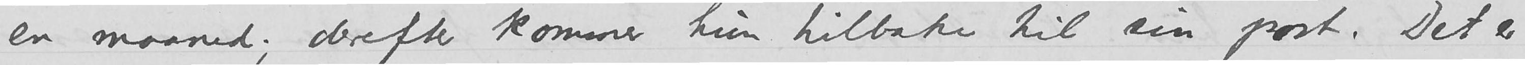en maaned; derefter kommer hun tilbake til sin post. Det er
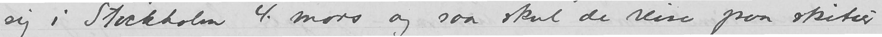sig i Stockholm 4. mars og saa skal de reise paa skitur
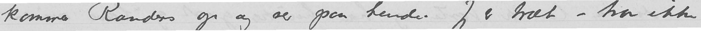kommer Randers op og ser på hende. Jeg er træt -tror ikke
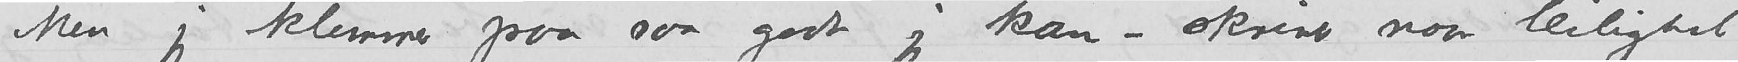Men jeg klemmer paa saa godt jeg kan – skriver naar leilighet
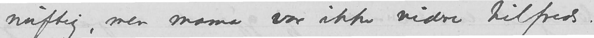nuftig, men mama var ikke videre tilfreds.
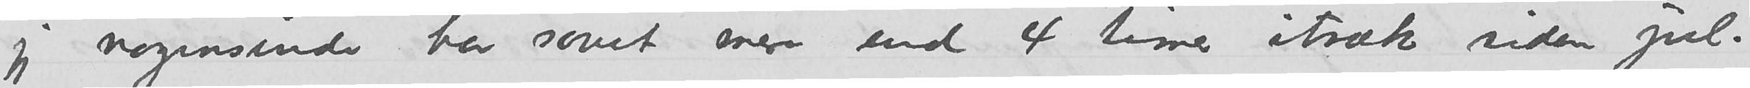jeg nogensinde har sovet mer end 4 timer itræk siden jul.
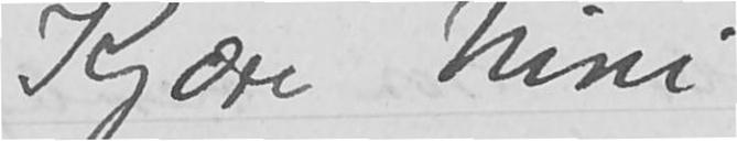Kjære Nini
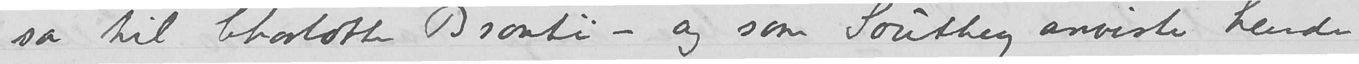sa til Charlotte Brontë -og som Southey anviste hende
